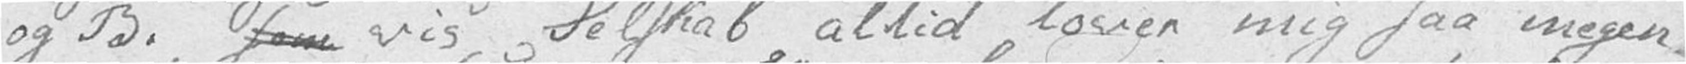og B. som vis Selskab altid lover mig saa megen
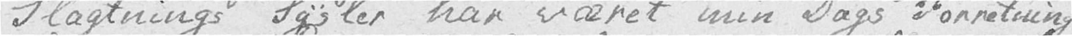Slagtnings Sysler har været min Dags Forretning
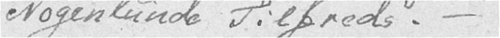Nogenlunde Tilfreds. –
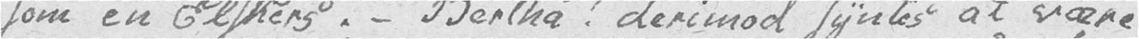som en Elskers. – Bertha! derimod syntes at være
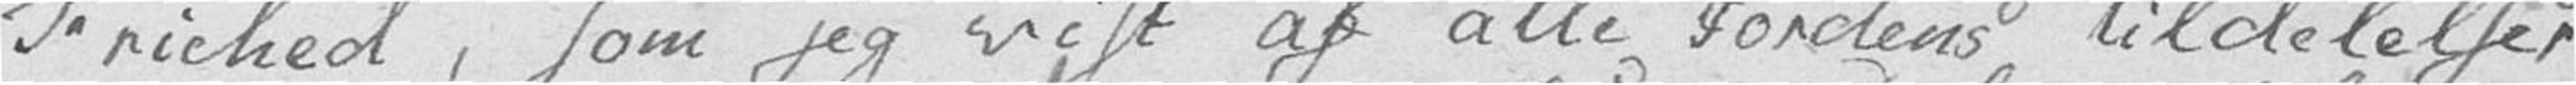Friehed, som jeg vist af alle Jordens tildelelser
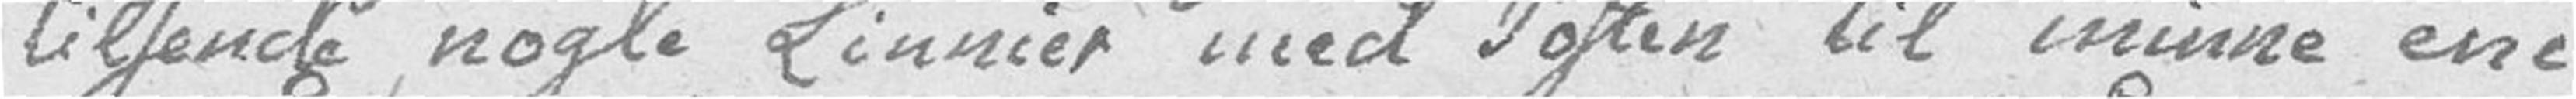tilsende nogle Linnier med Posten til minne ene
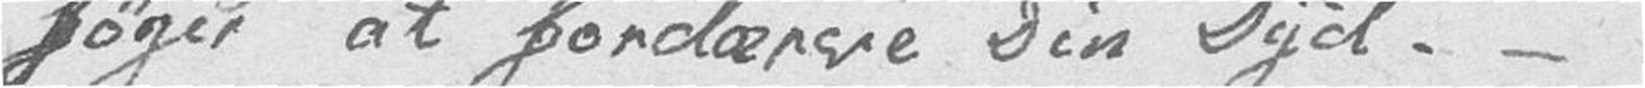søger at fordærve Din Dyd. –
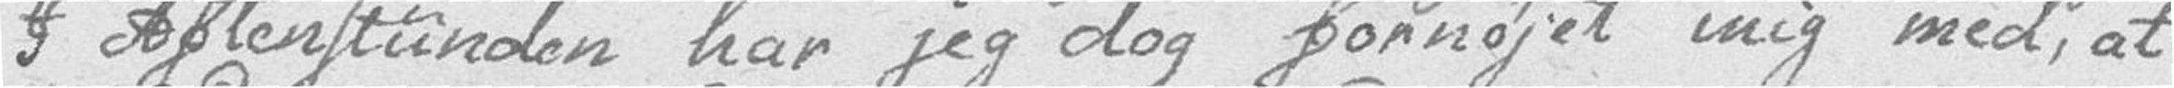I Aftenstunden har jeg dog fornøjet mig med, at
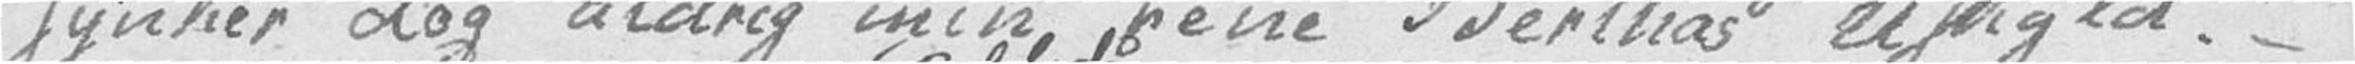synker dog aldrig min rene Berthas uskyld. –
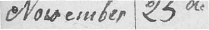November 25de
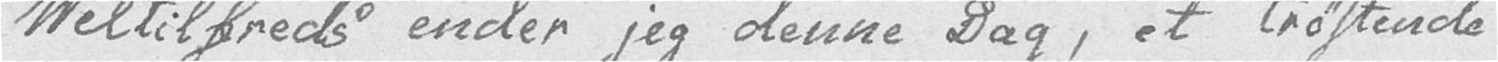Veltilfreds ender jeg denne Dag, et trøstende
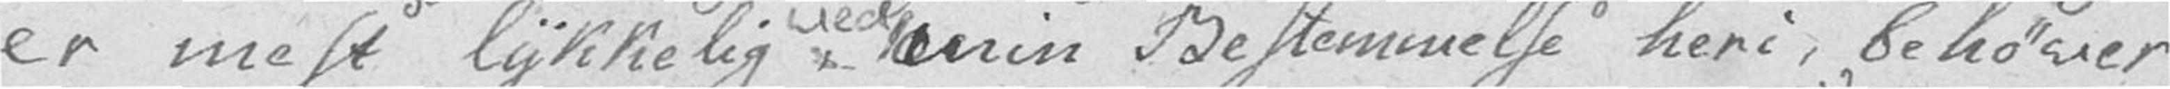er mest lykkelig ved min Bestemmelse heri, behøver
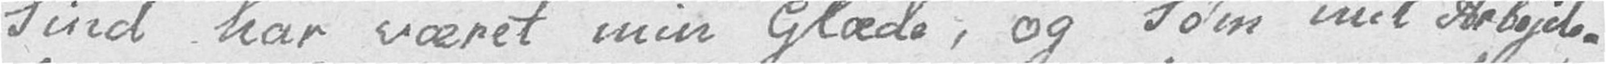Sind har været min Glæde, og Søm mit Arbejde.
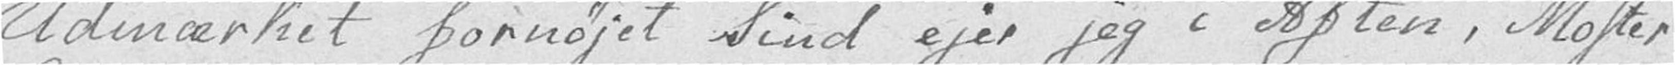Udmærket fornøjet Sind ejer jeg i Aften, Moster
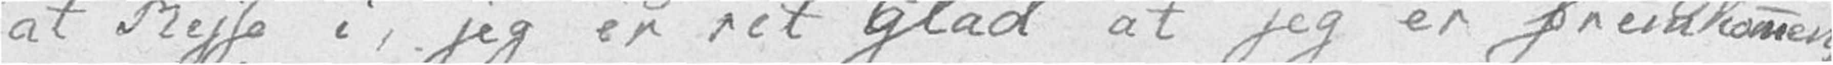at Rejse i, jeg er ret Glad at jeg er fremkommen
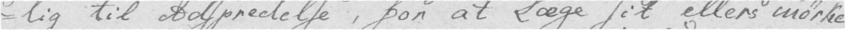-lig til Adspredelse, for at Læge sit ellers mørke
